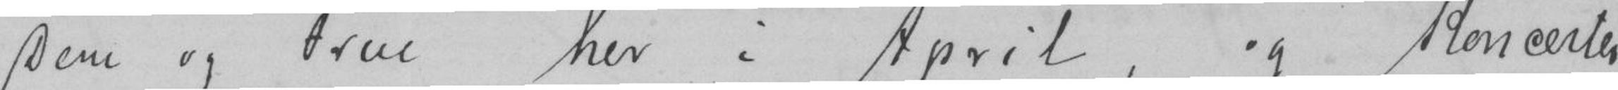Dem og Frue her i April, og Koncerten
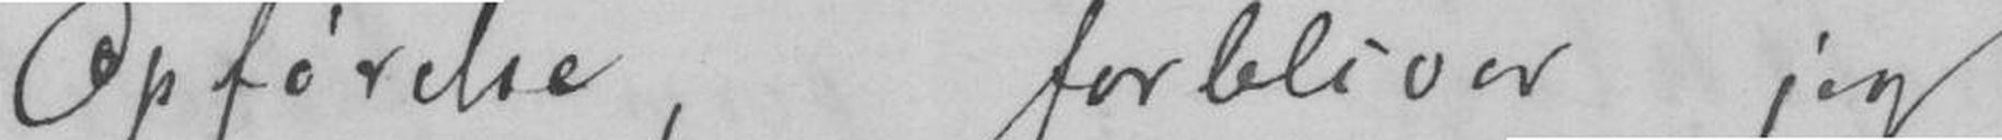Opførelse, forbliver jeg
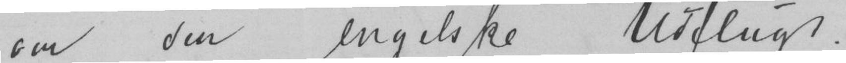om den engelske Udflugt.
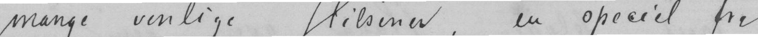mange venlige Hilsener, en opeciel fra
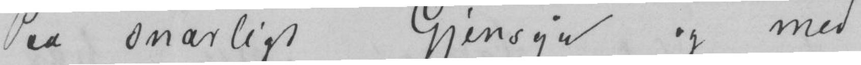Paa snarlig Gjensyn og med
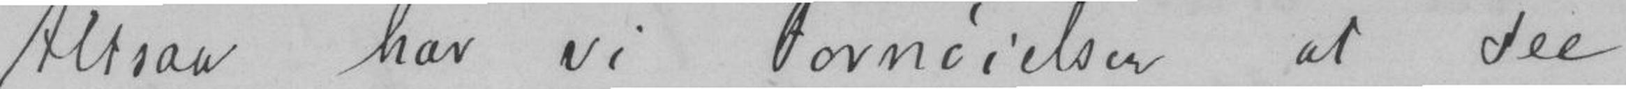Altsaa har vi Fornøielser at see
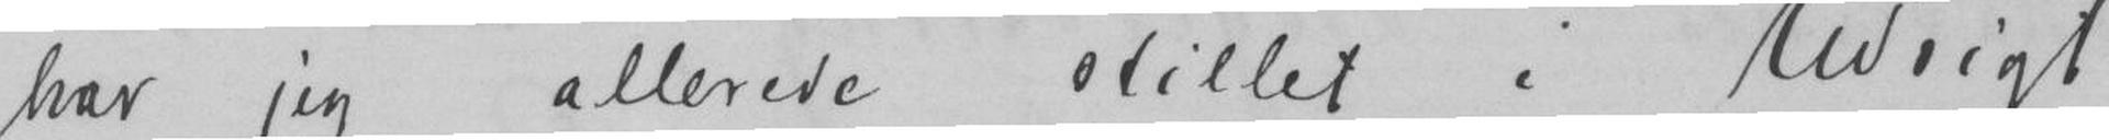har jeg allerede stillet i Udsigt
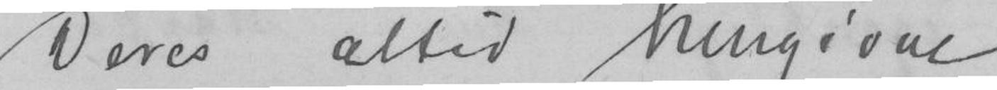Deres altid Hengivne
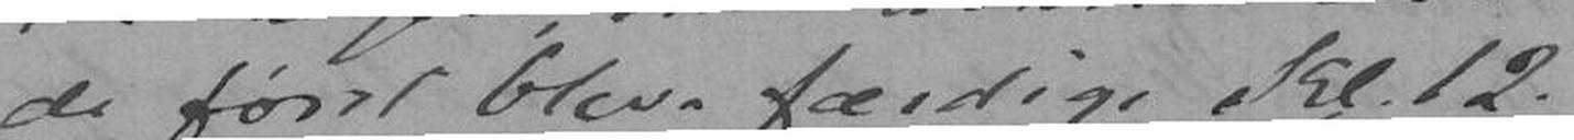de først bleve færdige Kl.12.
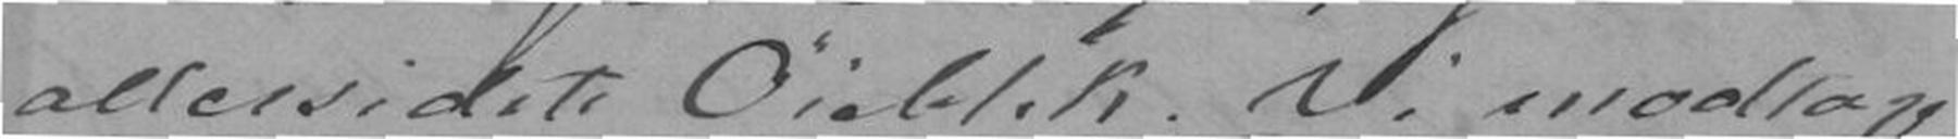allersidst Øieblik. Vi modtog
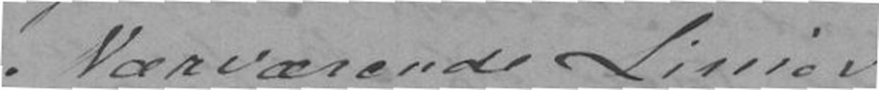Nærværende Linier
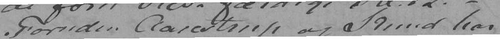Foruden Aarestrup og Knud har
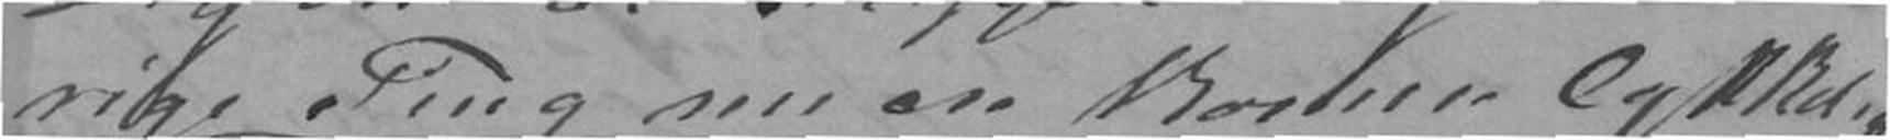rige Ting nu ere kommen lykkelig
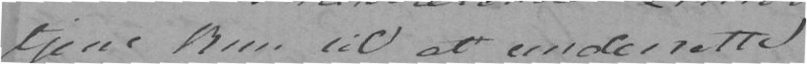tjene kun til at underrette
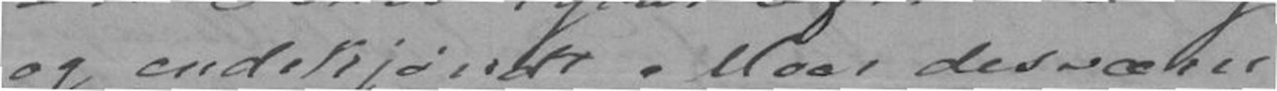og endskjøndt Moer desværre
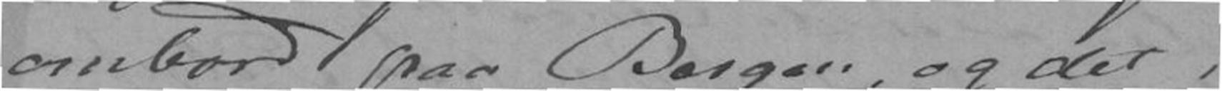ombord paa Bergen, og det i
